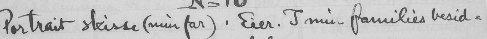Portrait skisse (min far), Eier. I min families besid-
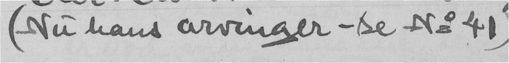(Nu hans arvinger - (se No 41)
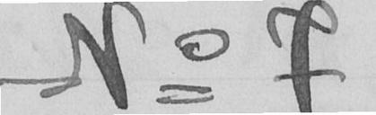No 7
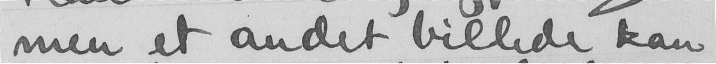men et andet billede kan
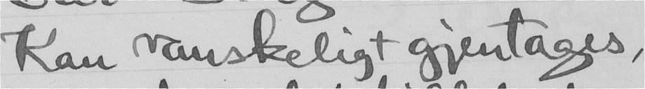Kan vanskeligt gjentages,
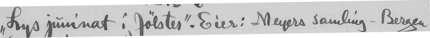"Lys juninat i Jølster". Eier: Meyers samling - Bergen
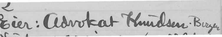Eier: Advokat Knudsen, Bergen
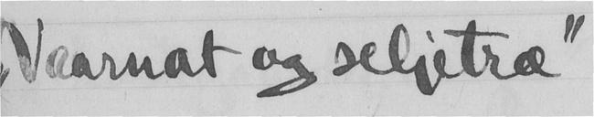"Vaarnat og seljetræ"
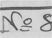No 8
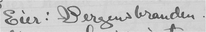Eier: i Bergens branden.
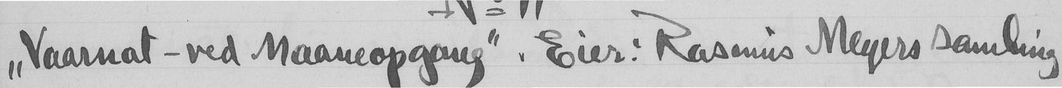"Vaarnat - ved "Maaneopgang", Eier: Rasmus Meyers samling
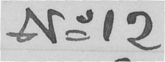No 12
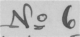No 6
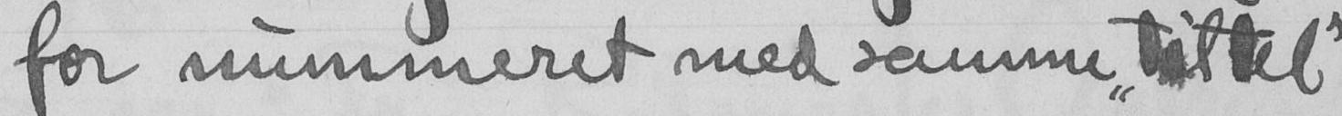for nummeret med samme "tittel"
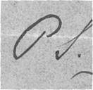PS.
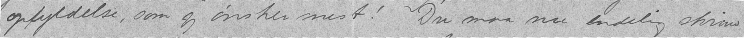opfyldelse, som jeg ønsker mest! Du maa nu endelig skrive
